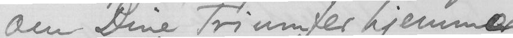om Dine Triumfer hjemme
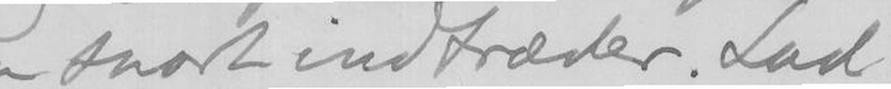som snart indtræder. Lad os
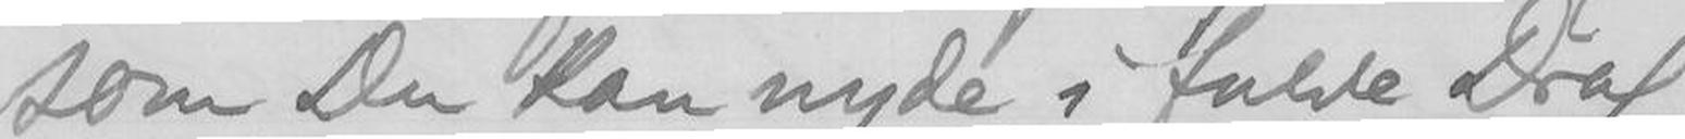som Du kan nyde i fulde Drag,
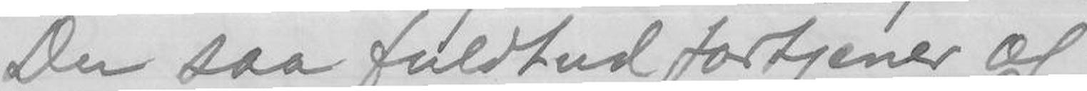Du saa fuldtud fortjener og
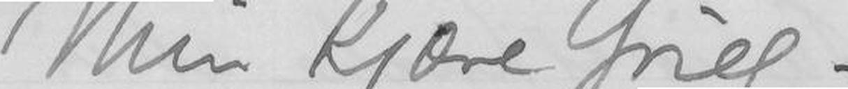Min kjære Grieg.
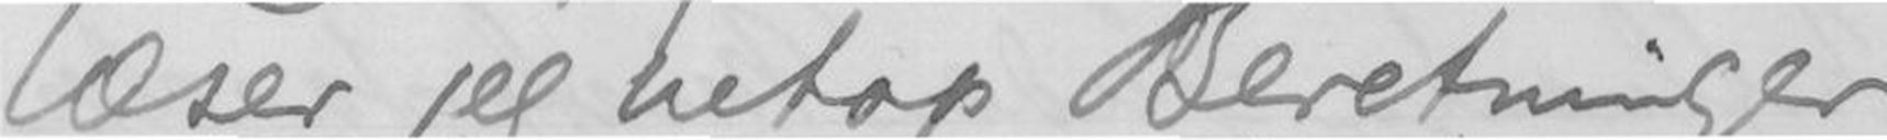læser jeg netop Beretninger
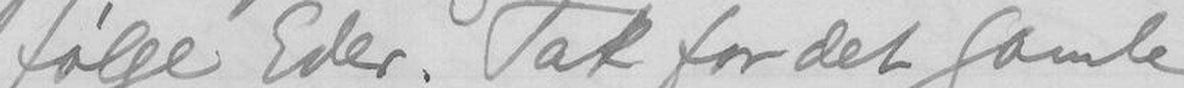følge Eder. Tak for det gamle
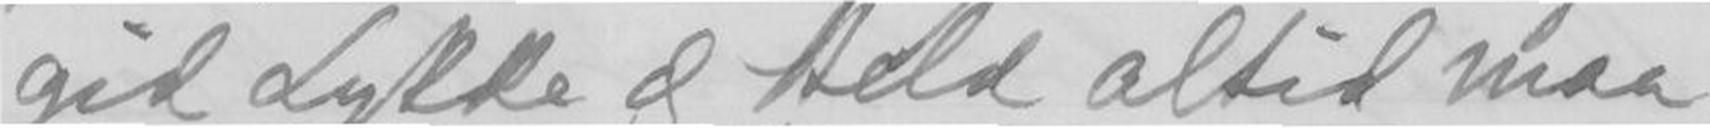gid Lykke og Held altid maa
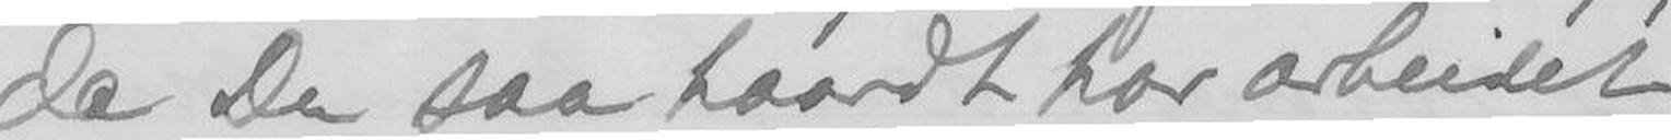da Du saa haardt har arbeidet
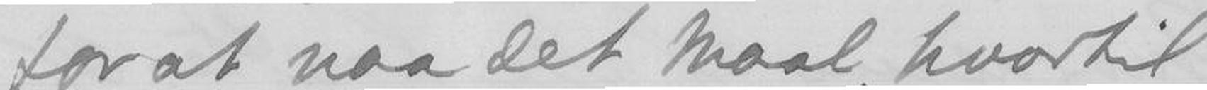for at naa det Maal hvortil
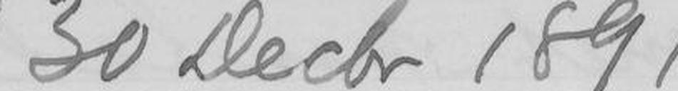30 Decbr 1891.
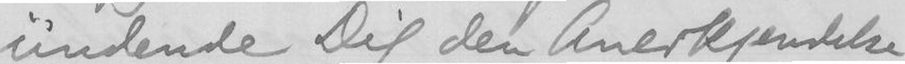undende Dig den Anerkjendelse
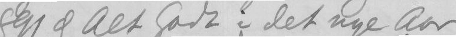1891 og Alt godt i det nye Aar,
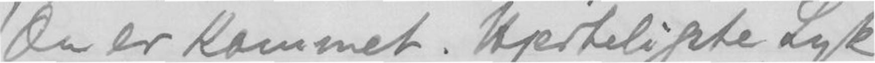Du er kommet. Hjerteligste Lyk-
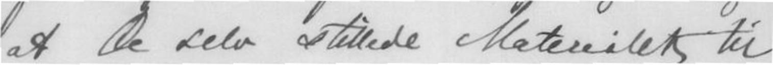at De selv stillede Materialet til
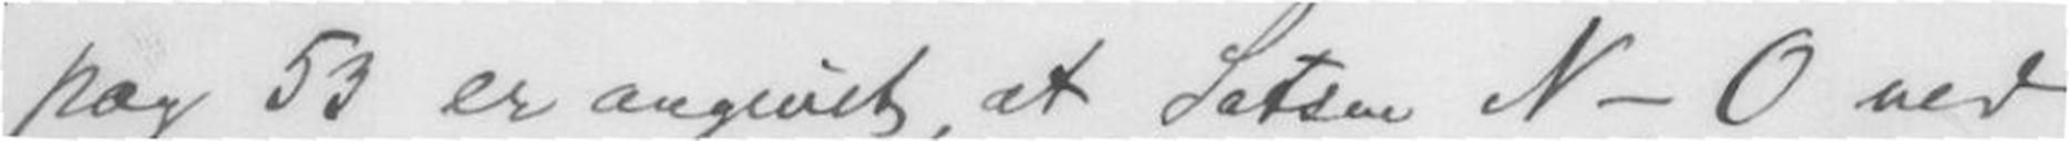pag 53 er angivet, at Satsen N - O med
